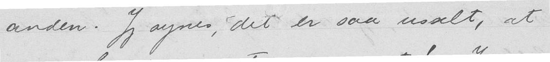anden. Jeg synes, det er saa usselt, at
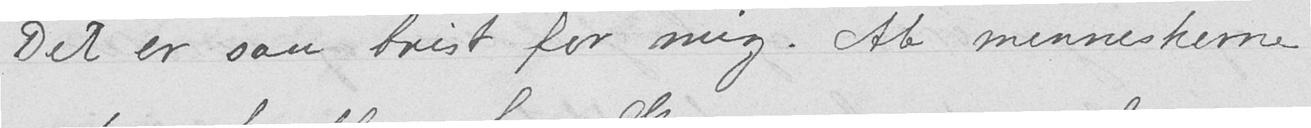Det er saa trist for mig. At menneskerne
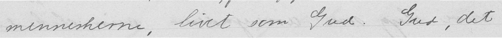menneskerne, livet som Gud. Gud, det
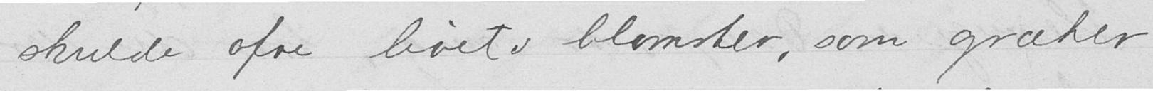skulde ofre livets blomster, som græker
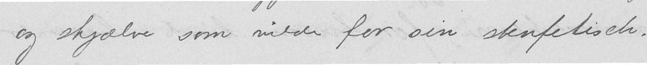og skjælve som vilde for sin stenfetisch.
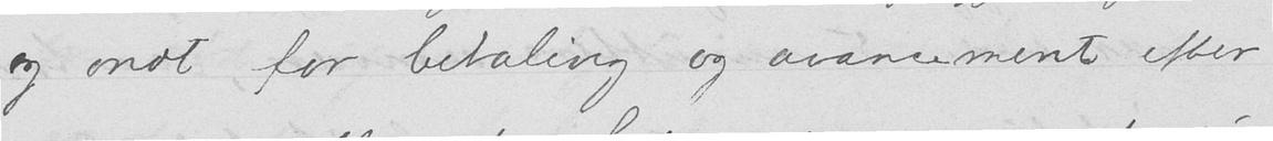og ondt for betaling og avancement efter
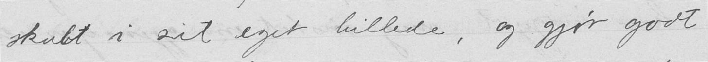skabt i sit eget billede, og gjør godt
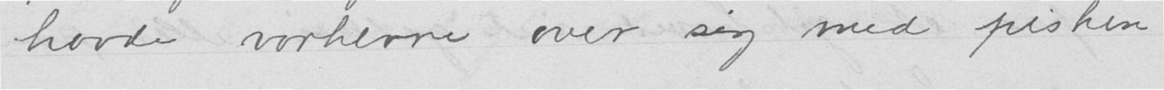havde vorherre over sig med pisken
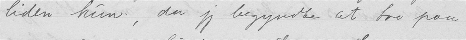liden kun, da jeg begyndte at tro paa
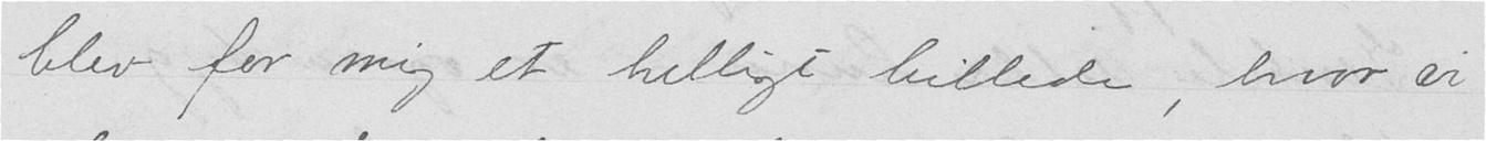blev for mig et helligt billede, hvor vi
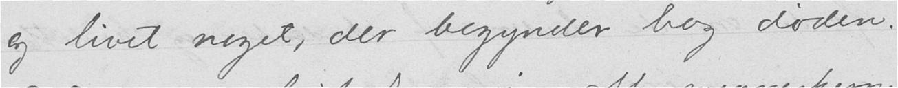og livet noget, der begynder bag døden.
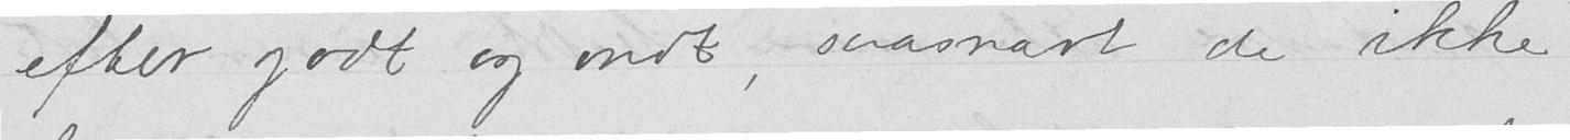efter godt og ondt, saasnart de ikke
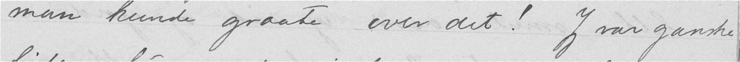man kunde graate over det! Jeg var ganske
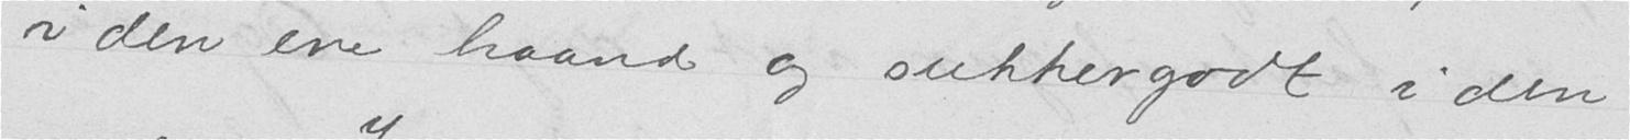i den ene haand og sukkergodt i den
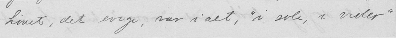Livet, det evige, var i alt, "i sole, i violer"
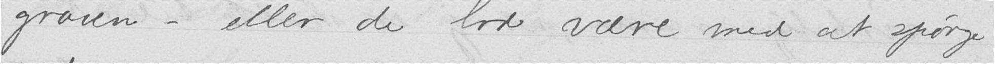graven - eller de lod være med at spørge
